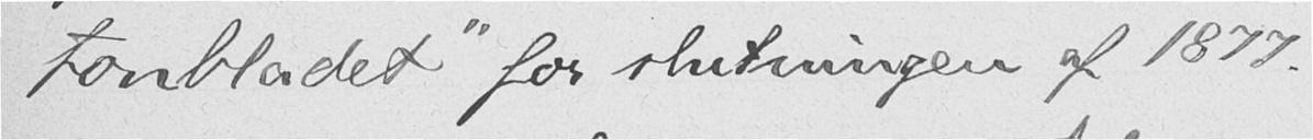tonbladet" for slutningen af 1877.
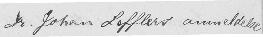Dr. Johan Lefflers anmeldelse
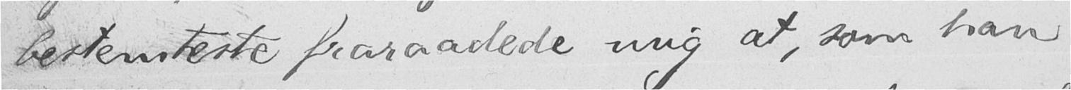bestemteste fraraadede mig at, som han
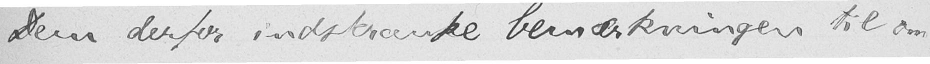Dem derfor indskrænke bemærkningen til om-
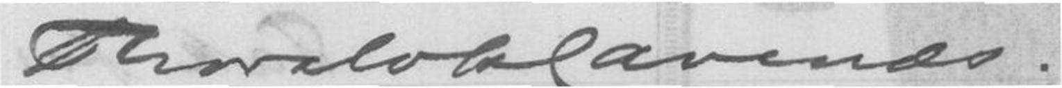Thorvald Klavenæs
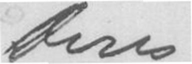Deres
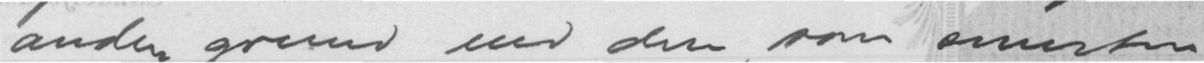anden grund end den, som smerten
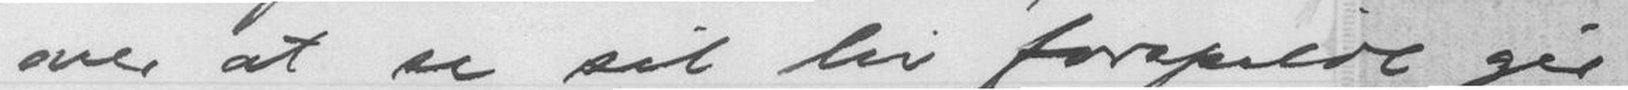over at se sit liv forespilde gir.
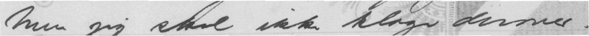Men jeg skal ikke klage dermed.
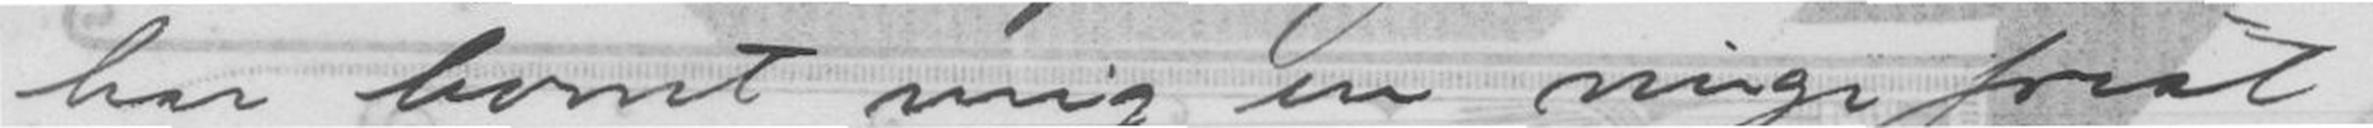har komt mig en mager frist.
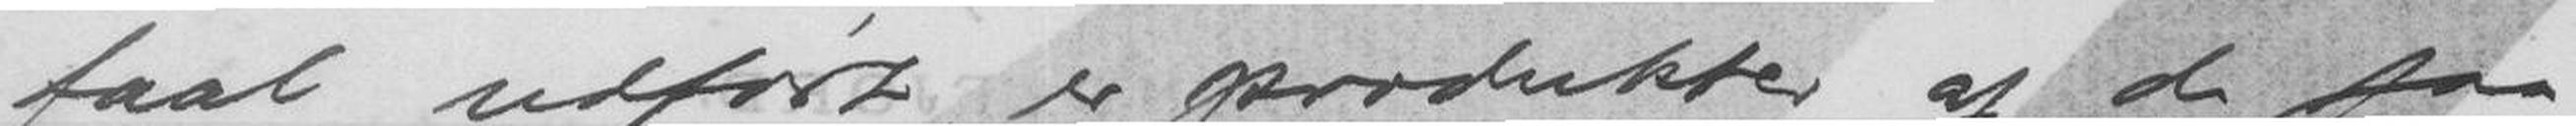faaet udført, er produkter af de faa
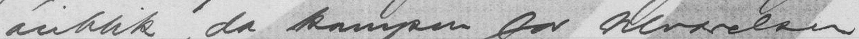øieblik, da kampen for tilværelsen
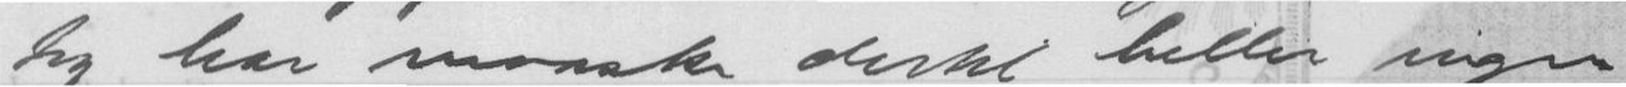Jeg har maaske dertil heller ingen
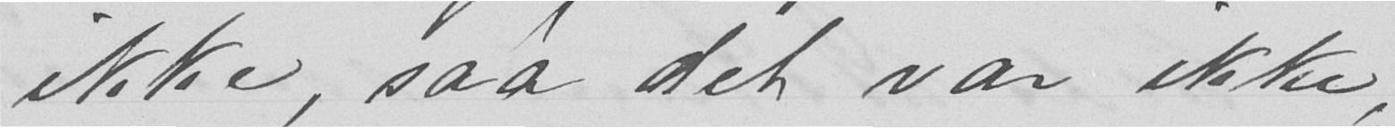ikke, saa det var ikke,
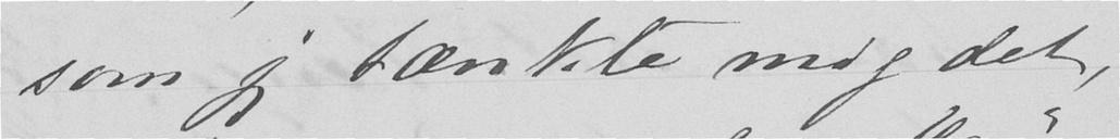som jeg tænkte mig det,
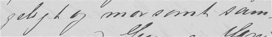geligt og morsomt sam-
